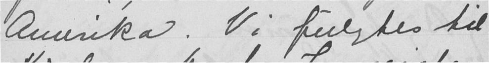Amerika. Vi fulgtes til
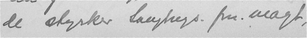de styrker Saugbrugs. frn. magt,
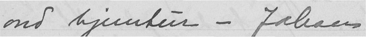ond hjemtur - Johan
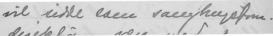vil sidde som saugbrugsforn.
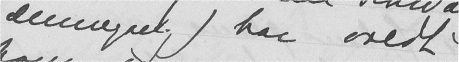dennegang) har voldt
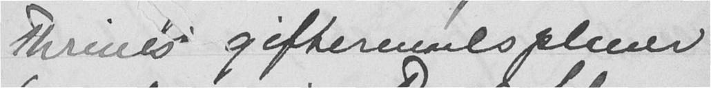Thrines giftermaalsplaner
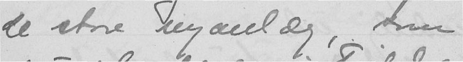de store nyanlæg, som
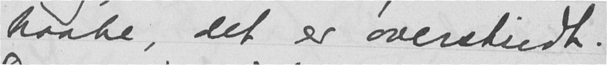haabe, det er overstiedt.
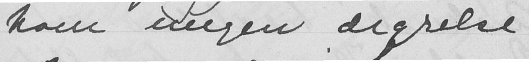ham ingen ærgrelse
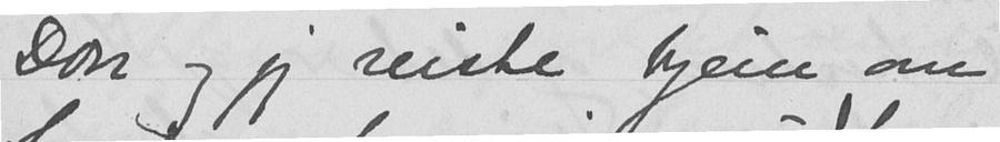Don og jeg reiste hjem om
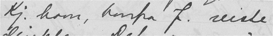Kj. havn, hvorfra J. reiste
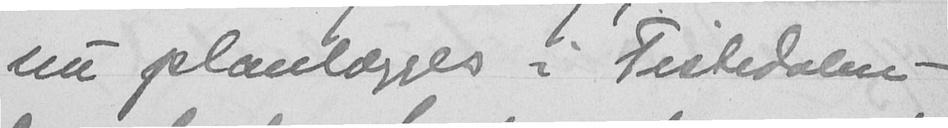nu planlægges i Tistedalen -
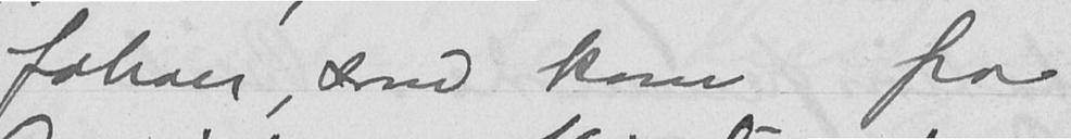Johan, som kom fra
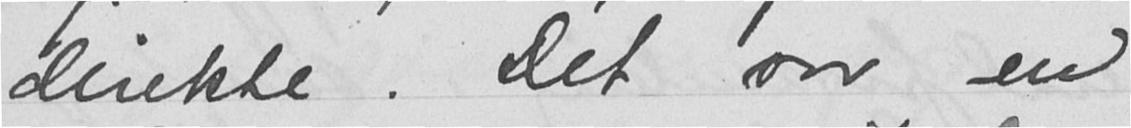direkte. Det var en
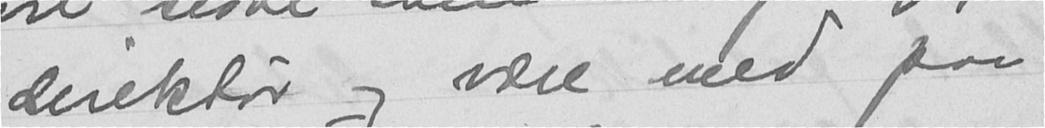direktør og være med paa
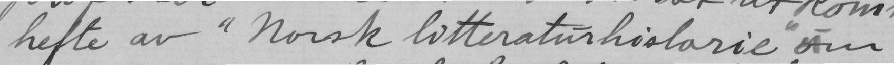hefte av "Norsk litteraturhistorie" om
